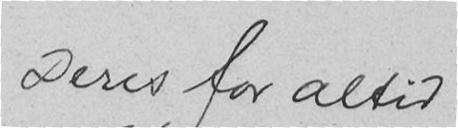Deres for altid
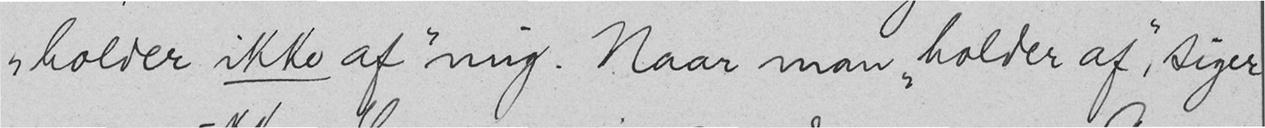"holder ikke af" mig. Naar man "holder af", siger
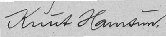Knut Hamsun.
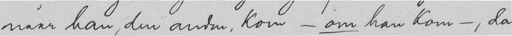naar han, den anden, kom - om han kom -, da
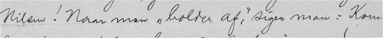Nilsen! Naar man "holder af", siger man: Kom
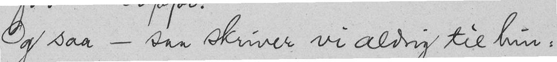Og saa - saa skriver vi aldrig til hin-
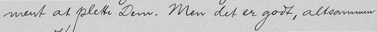ment at plette Dem. Men det er godt, altsammen
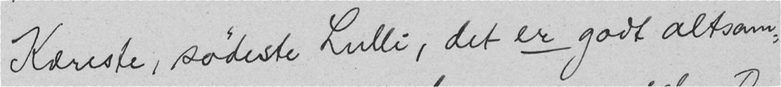Kæreste, sødeste Lulli, det er godt altsam-
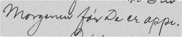Morgenen før De er oppe.
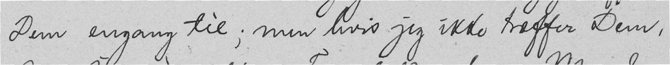Dem engang til; men hvis jeg ikke træffer Dem,
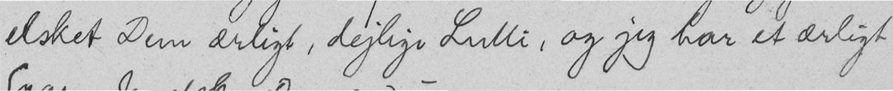elsket Dem ærligt, dejlige Lulli, og jeg har et ærligt
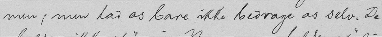men; men lad os bare ikke bedrage os selv. De
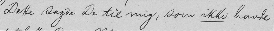Dette sagde De til mig, som ikke havde
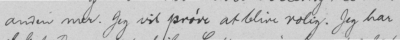anden mer. Jeg vil prøve at blive rolig. Jeg har
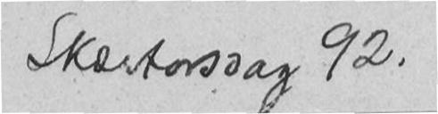Skærtorsdag 92.
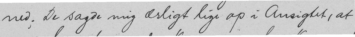ned. De sagde mig ærligt lige op i Ansigtet, at
